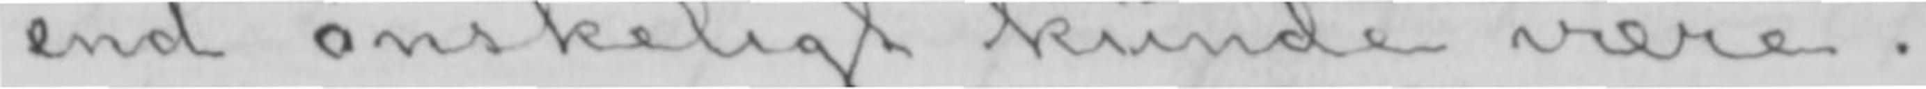end ønskeligt kunde være.
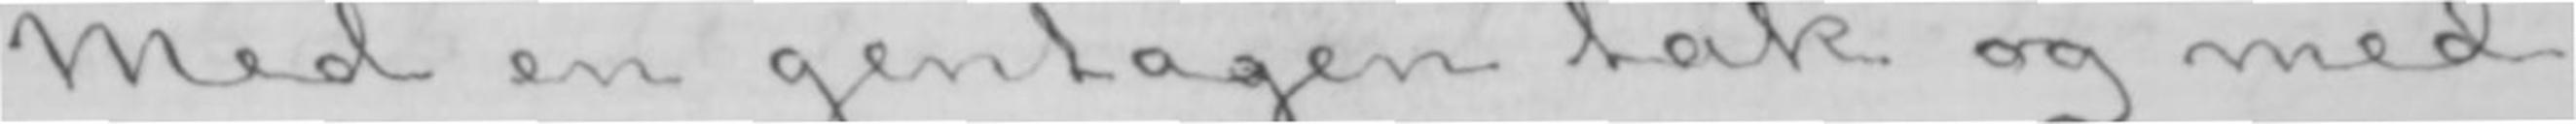Med en gentagen tak og med
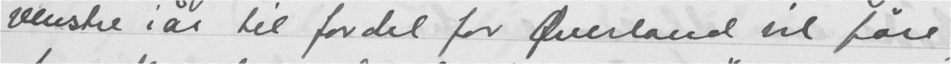venstre iår til fordel for Øverland vil føre
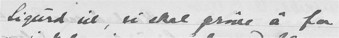Sigurd vil, vi skal prøve å få
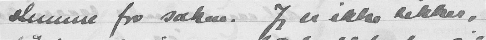stemme for saken. Jeg er ikke sikker,
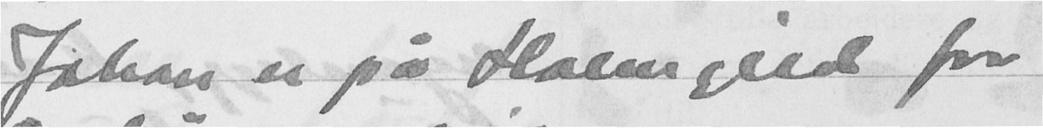Johan er på Holmgild for
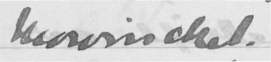Mowinckel.
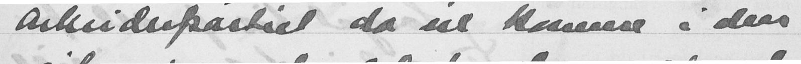arbeiderpartiet da vil komme i den
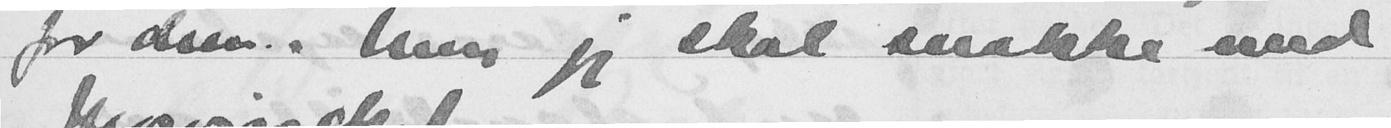for dem. Men jeg skal snakke med
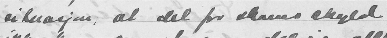situasjon, at det for skams skyld
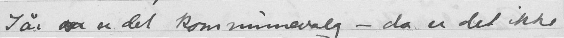"Så  er det kommunevalg - da er det ikke
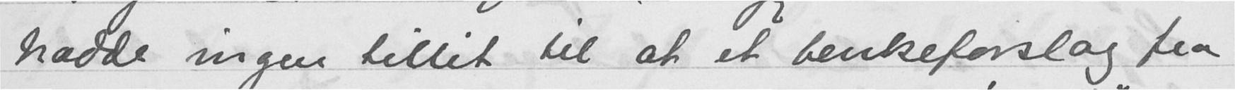hadde ingen tillit til at et benkeforslag fra
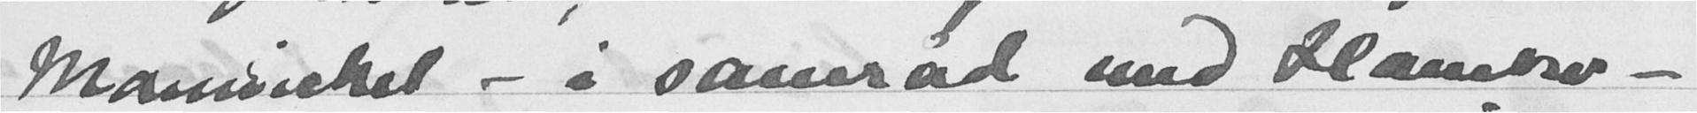Mowinckel - i samråd med Hambro -
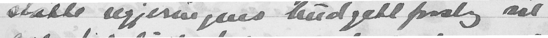støtte regjeringens budsjettforslag vil
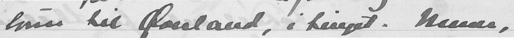lønn til Øverland, i tinget. Mener,
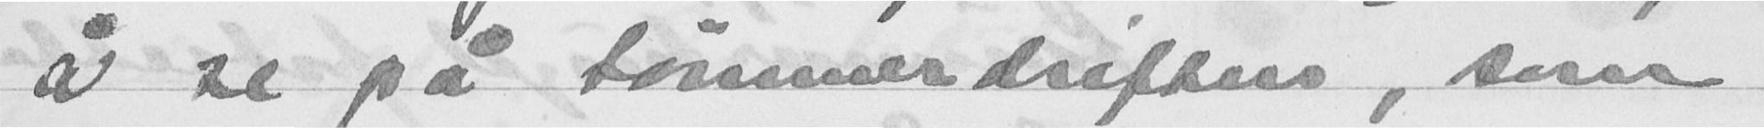å se på tømmerdriften, som
